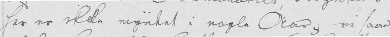her er ikke myntet i nogle Aar; vi saae
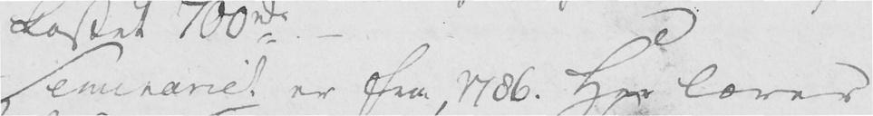Seminariet er fra 1786. Her lærer
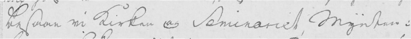besaae vi Kirken og Seminariet, Mynten i
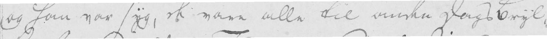og han var syg, de vare alle til anden Dags Bryl-
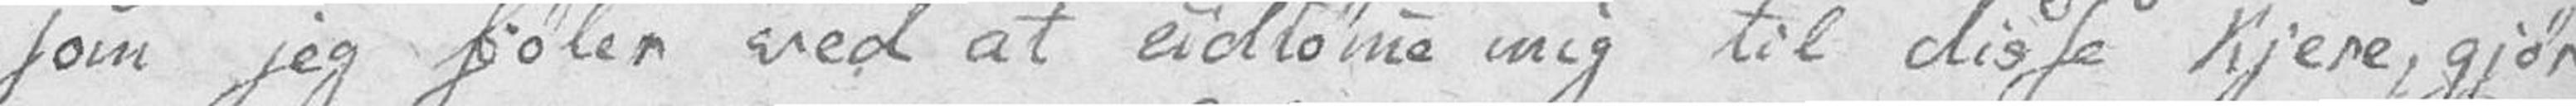som jeg føler ved at udtømme mig til disse kjere, gjør
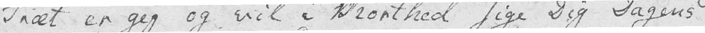Træt er jeg og vil i Korthed sige Dig Dagens
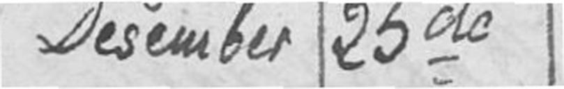Desember 25de
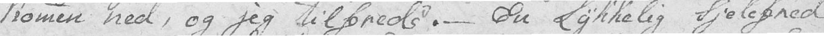kommen ned, og jeg tilfreds. – En Lykkelig Sjelefred
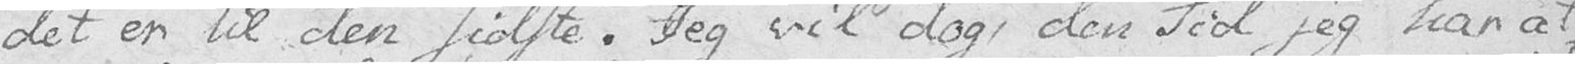det er til den sidste. Jeg vil dog, den Tid jeg har at
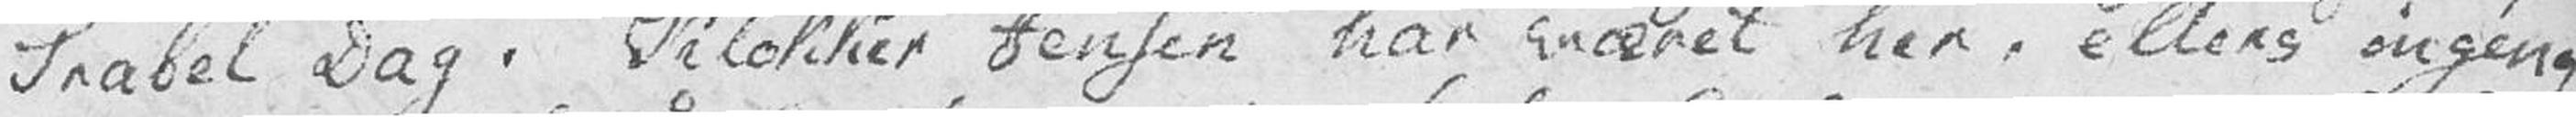Trabel Dag. Klokker Jensen har været her, ellers ingen.
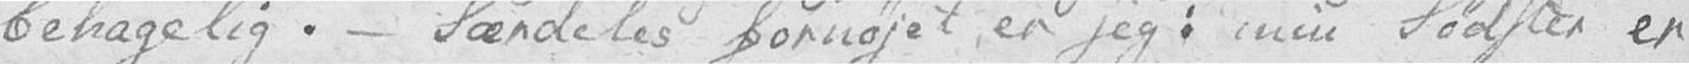behagelig. – Særdeles fornøjet er jeg: min Sødster er
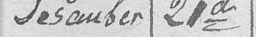Desember 21de
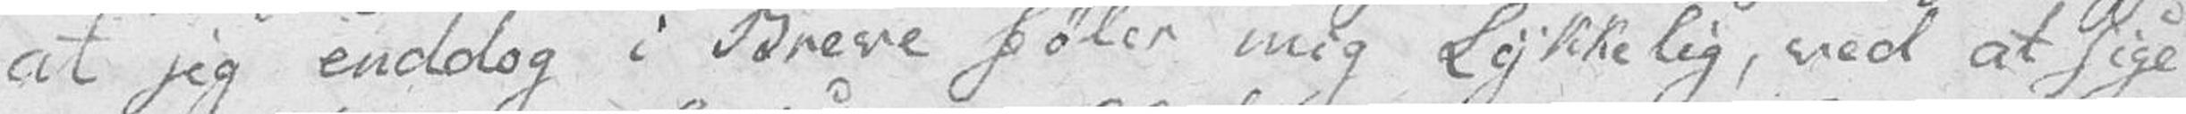at jeg enddog i Breve føler mig Lykkelig, ved at sige
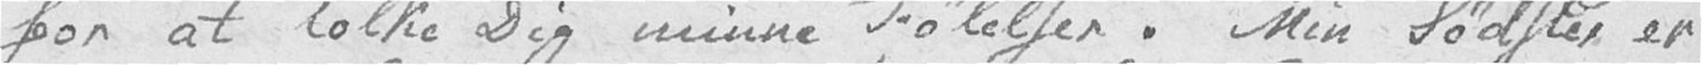for at tolke Dig minne Følelser. Min Sødster er
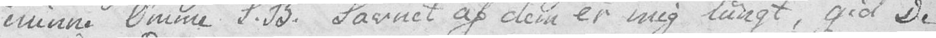minne Ømme S. B. Savnet af dem er mig tungt, gid De
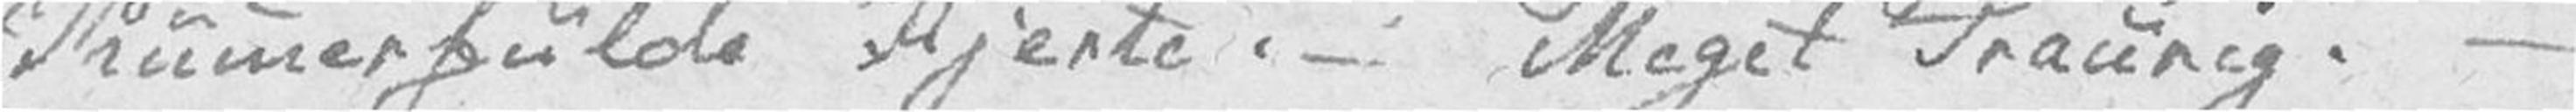Kummerfulde Hjerte. – Meget Traurig. –
